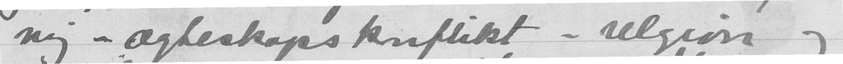mig - ægteskapskonflikt - relgion og
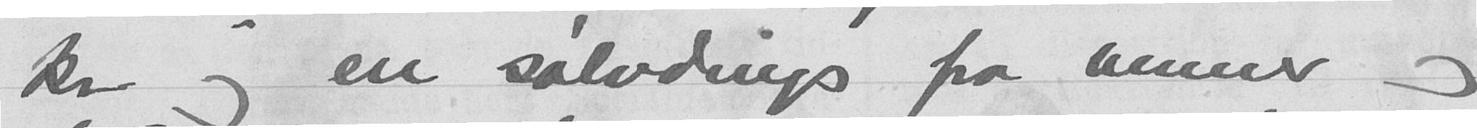kr. og en sølvdings fra venner og
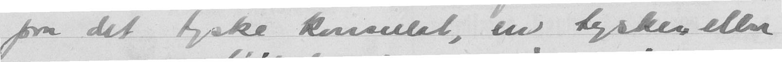paa det tyske konsulat, en tysker eller
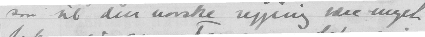saa vil den norske regjering være meget
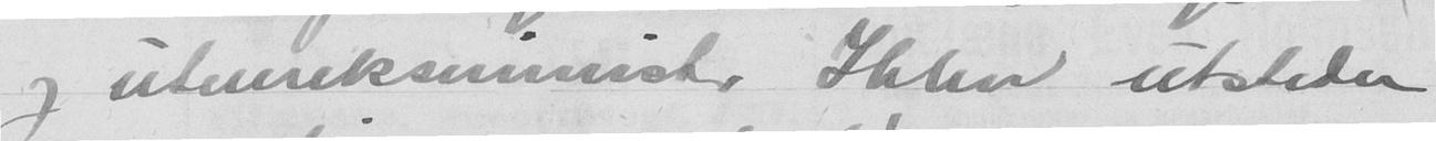og utenriksminister Ihlen utsteder
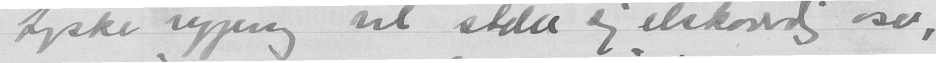tyske regjering vil stille sig elskværdig over,
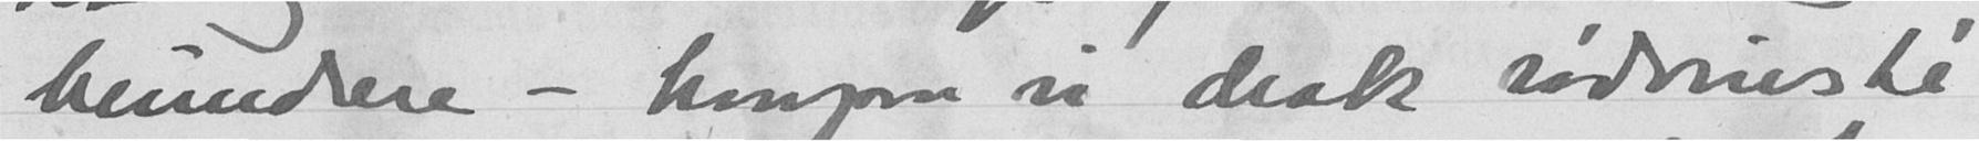beundrere - hvorpaa vi drak rødvinsté
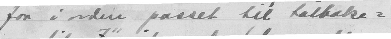faa i orden passet til tilbake-
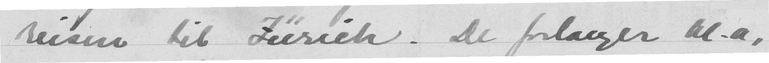reisen til Zürich. De forlanger bl. a,
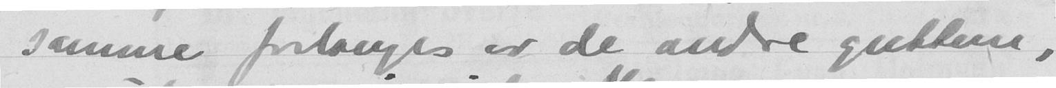samme forlanges av de andre guttene,
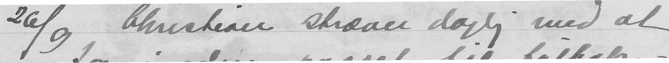26/9 Christian stræver daglig med at
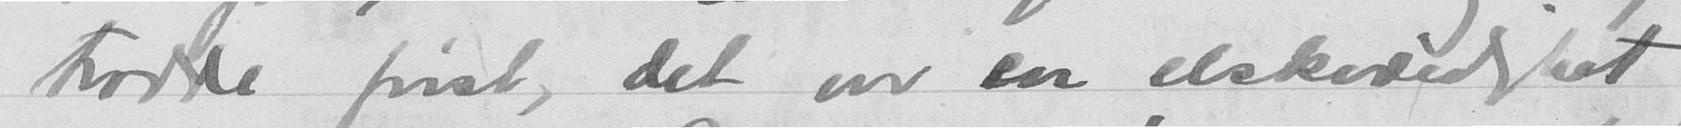trodde først, det var en elskvældighet
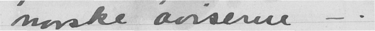norske aviserne - .
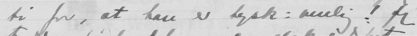ti for, at han er tysk-venlig! Jeg
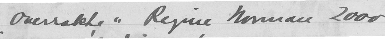"Overrakte" Regine Norman 2000
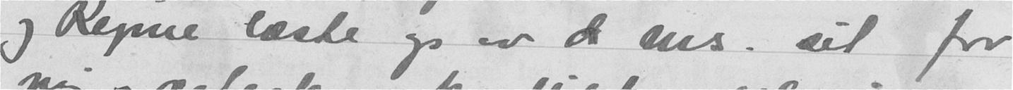og Regine læste op av d sns. sit for
